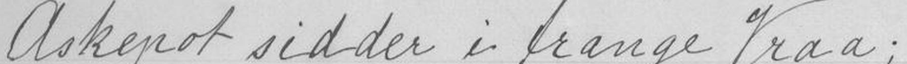Askepot sidder i trange Vraa;
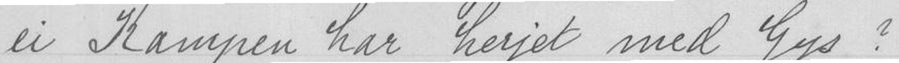ei Kampen har herjet med Gys?
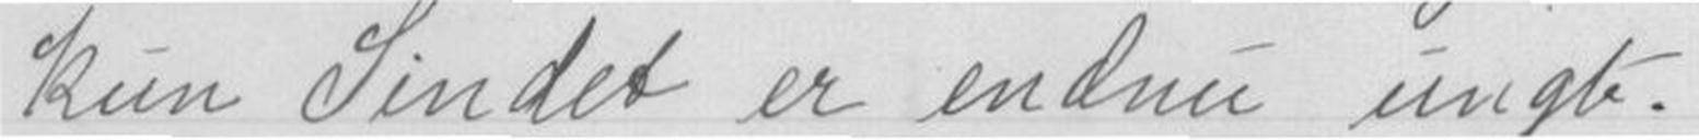kun Sindet er endnu ungt.
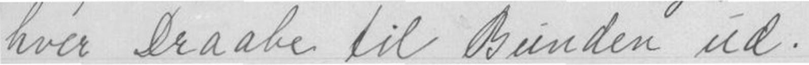hver Draabe til Bunden ud.
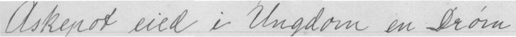Askepot eied i Ungdom en Drøm
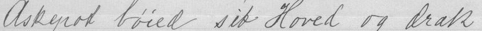Askepot bøied sit Hoved og drak
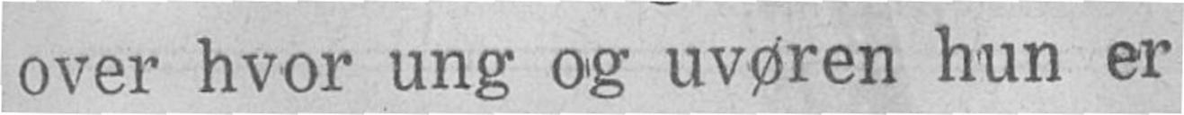over hvor ung og uvøren hun er
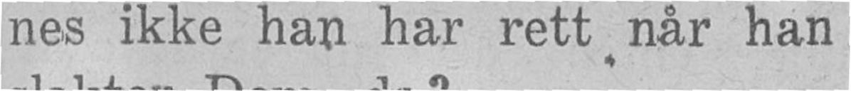nes ikke han har rett når han
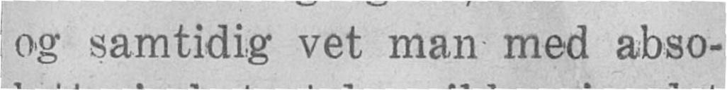og samtidig vet man med abso-
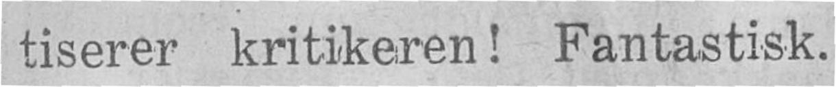tiserer kritikeren! Fantastisk.
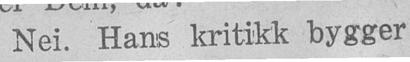- Nei. Hans kritikk bygger
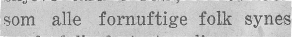som alle fornuftige folk synes
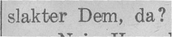slakter Dem, da?
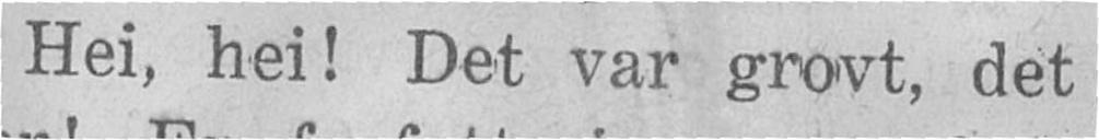Hei, hei! Det var grovt, det
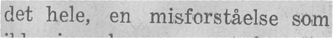det hele, en misforståelse som
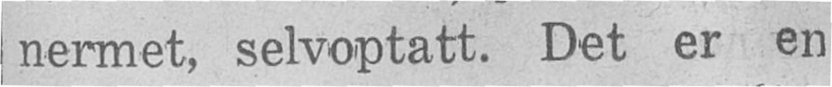nermet, selvoptatt. Det er en
